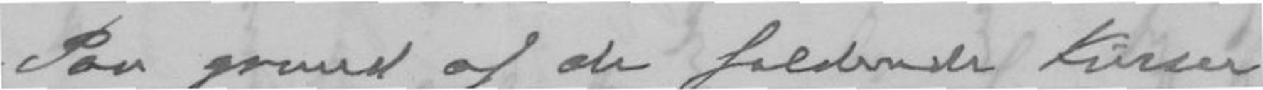Paa grund af de faldende kurser
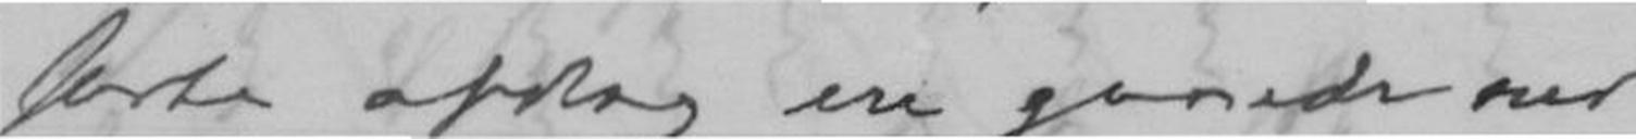førte afdrag ere gaaede ned
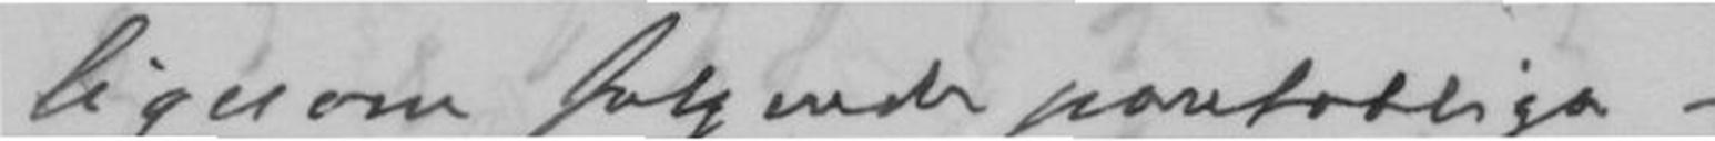ligesom følgende pantobliga-
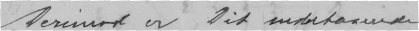Derimod er Dit indestaaende
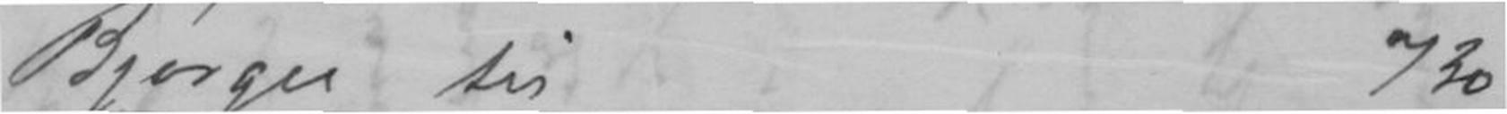Bjørges til 730
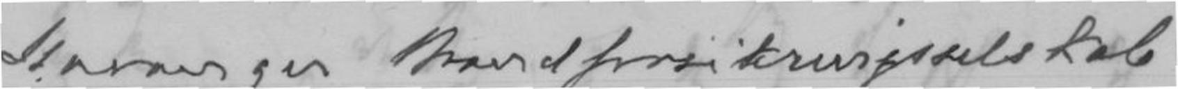Stavanger Brandforsikringsselskab
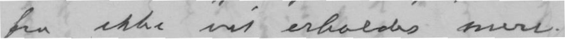for ikke vil erholdes mere.
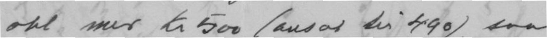obl med kr 500 (ansat til 590), saa
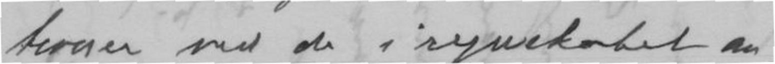tioner ved de i regnskabet an-
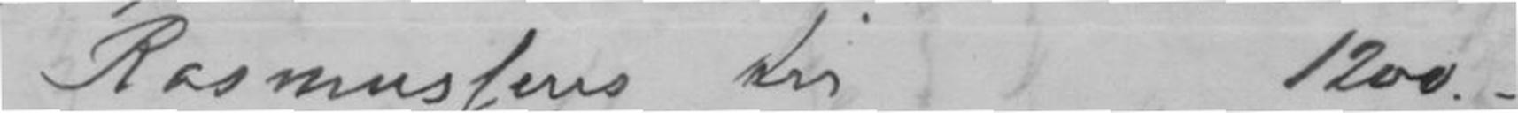og Rasmussens til1200.-
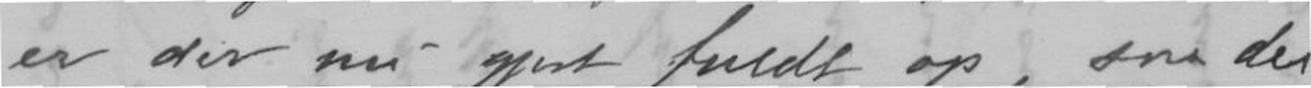er der nu gjort fuldt op, saa der-
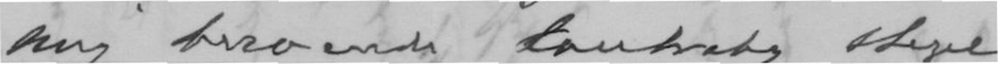mig beroende kontrabog stege
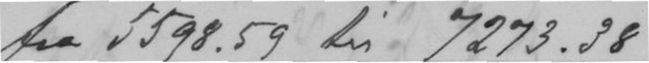fra 5598.59 til 7273.38
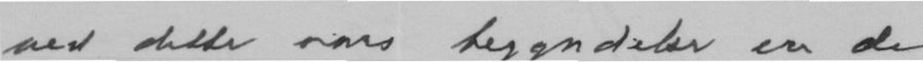ved dette aars begyndelse er de
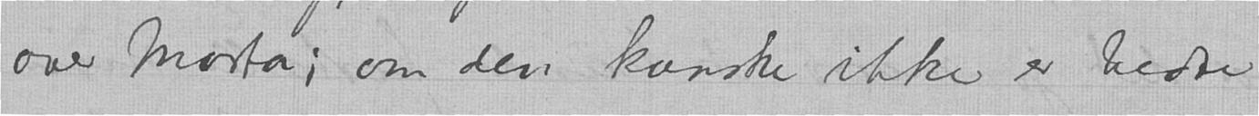over Marta; om den kanske ikke er bedre
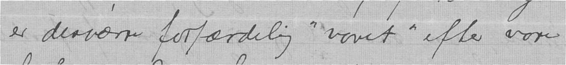er desværre forfærdelig "vovet" efter vore
